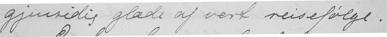gjensidig glæde af vort reisefølge.
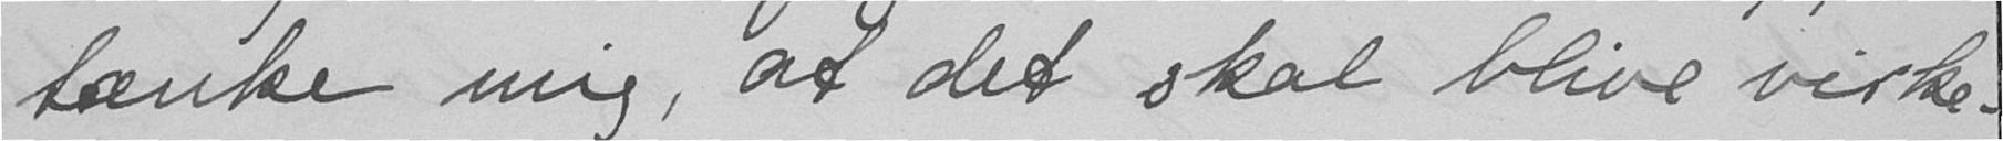tænke mig, at det skal blive virke
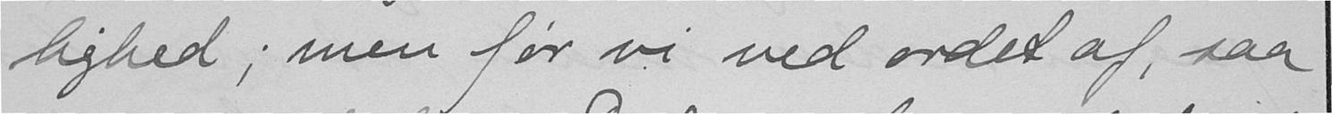lighed; men før vi ved ordet af saa
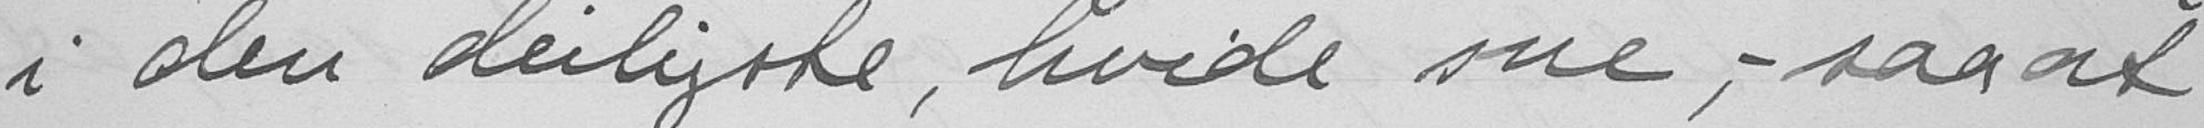i den delligste, hvide sne, - saa at
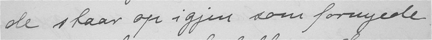de staar op igjen som fornyede
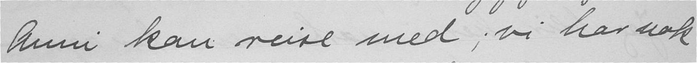Anni kan reise med; vi har nok
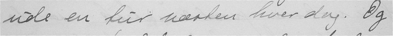ude en tur næsten hver dag. Og
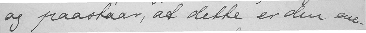og paastaar, at dette er den en-
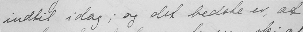indtil idag; og det bedste er, at
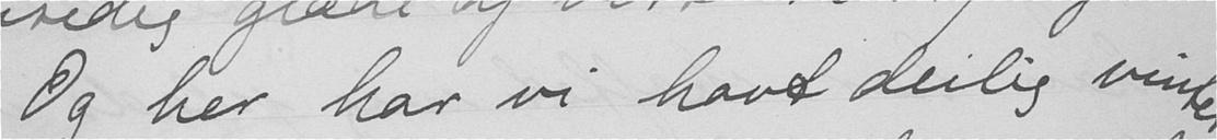Og her har vi havt deilig vinter
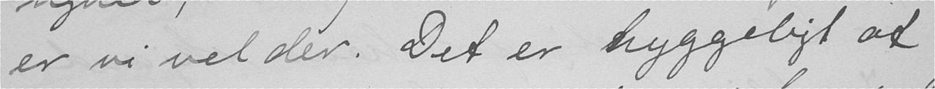er vi vel der. Det er hyggeligt at
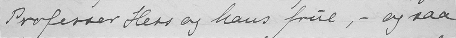Professor Hers og hans frue, - og saa
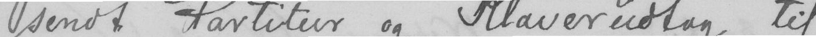sendt Partitur og Klaverudtag til
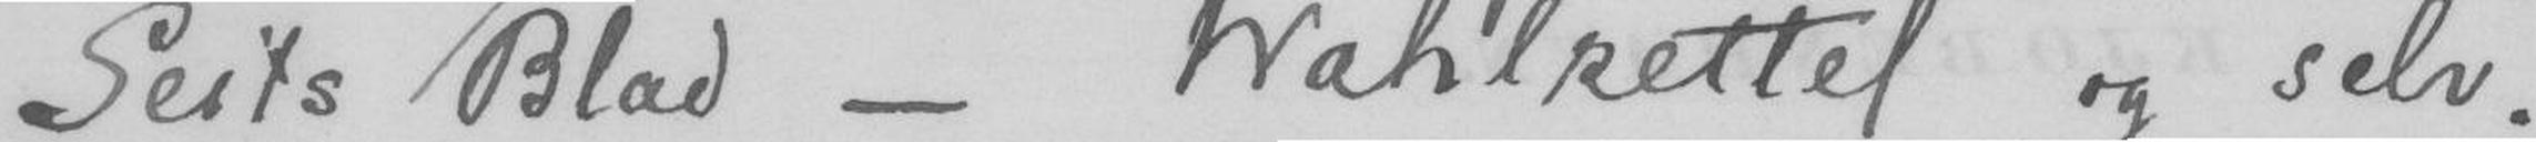Seits Blad - Wahlrettel og selv
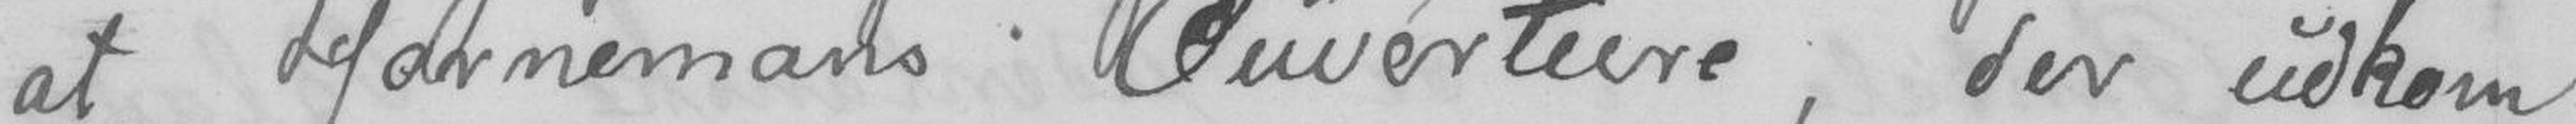at Hornemans Ouverture, der udkom
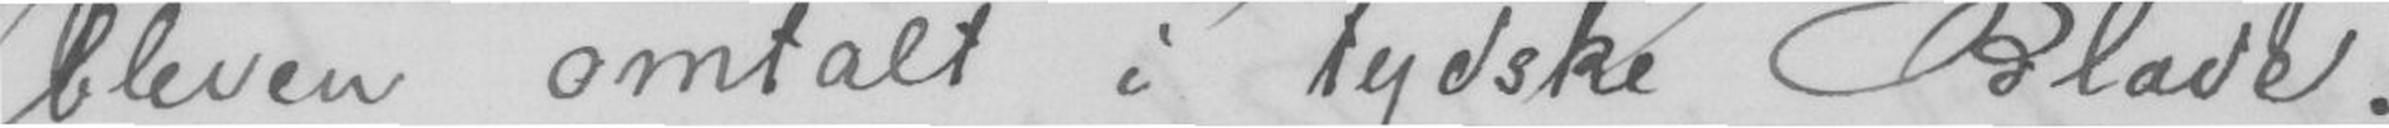bleven omtalt i tydske Blade.
